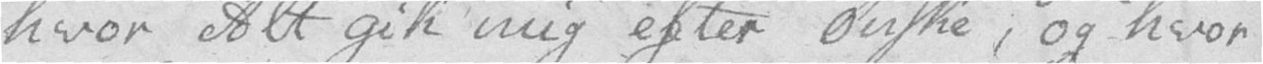hvor Alt gik mig efter Ønske, og hvor
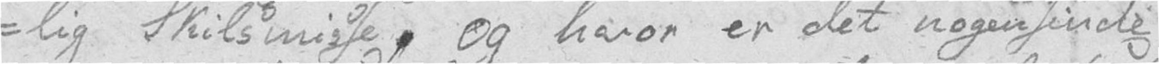-lig Skilsmisse, og hvor er det nogensinde
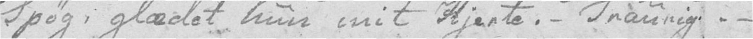Spøg, glædet hun mit Hjerte. – Traurig. –
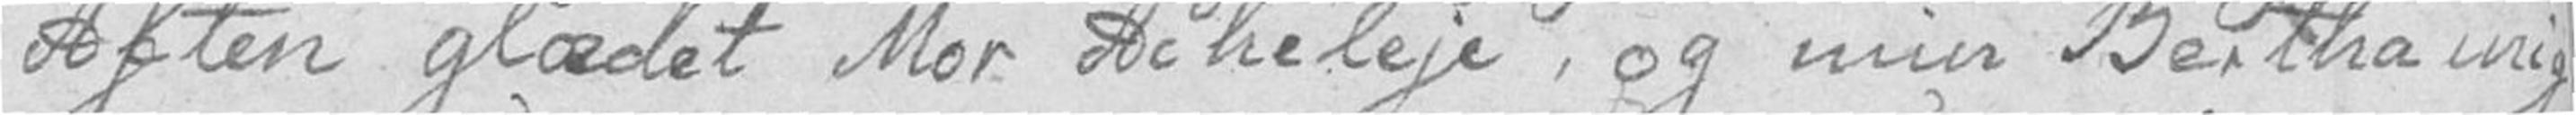Aften glædet Mor Acheleje, og min Bertha mig
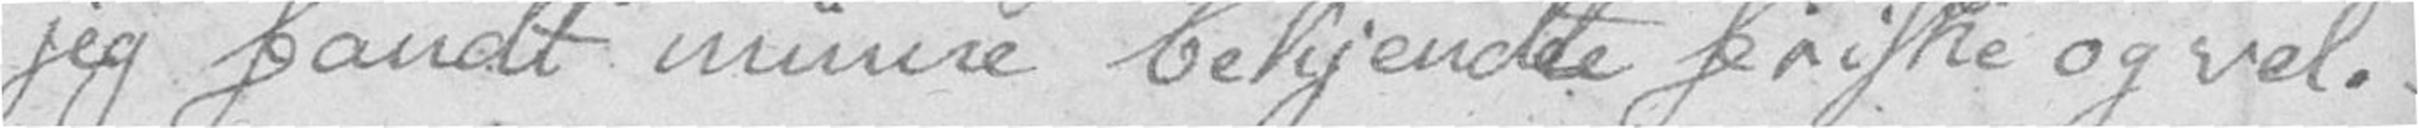jeg fandt minne bekjendte friske og vel.
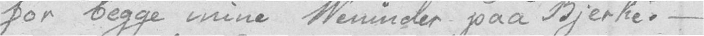for begge mine Veninder paa Bjerke. –
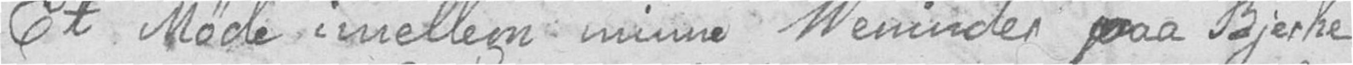Et Møde imellem minne Veninder paa Bjerke
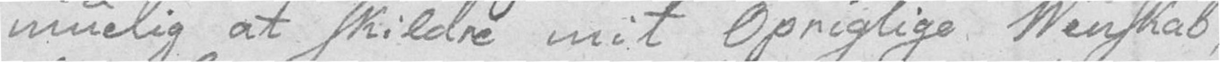muelig at skildre mit Oprigtige Venskab,
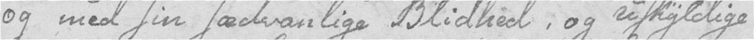og med sin sædvanlige Blidhed, og uskyldige
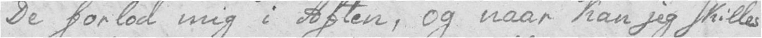De forlod mig i Aften, og naar kan jeg skilles
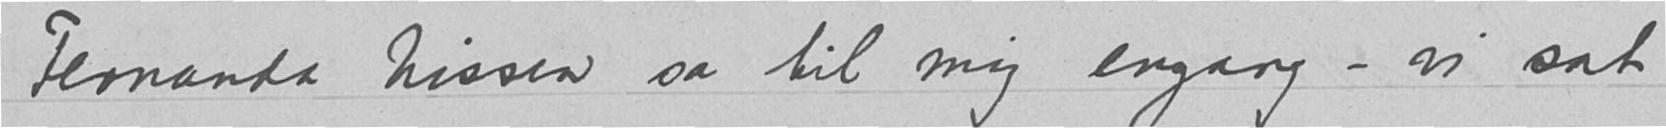Fernanda Nissen sa til mig engang - vi sat
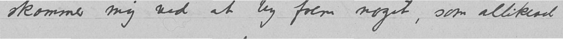skammer mig ved at by frem noget, som allikevel
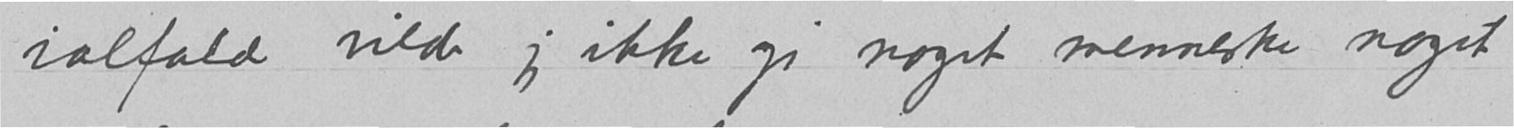ialfald vilde jeg ikke gi noget menneske noget
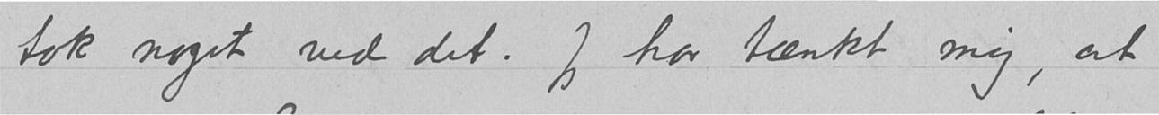tok noget ved det. Jeg har tænkt mig, at
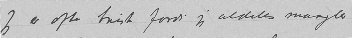Jeg er ofte trist fordi jeg aldeles mangler
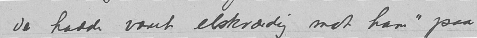De hadde været elskværdig mot ham "paa
